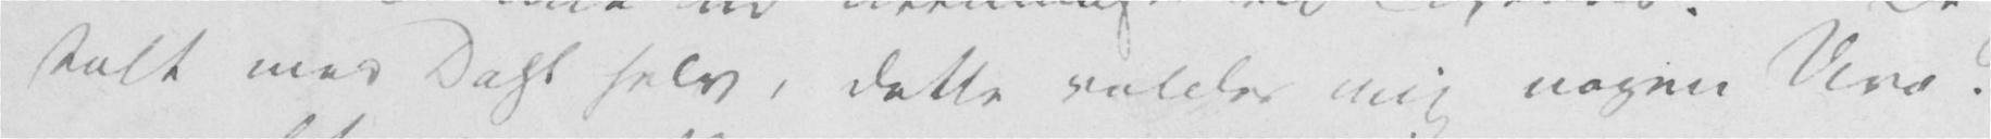talt med Dahl selv, dette volder mig nogen Uro.
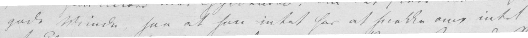gode Minde, saa at han intet har at snakke om, intet
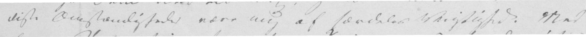disse Omstændigheder være mig af særdeles Vigtighed. Med
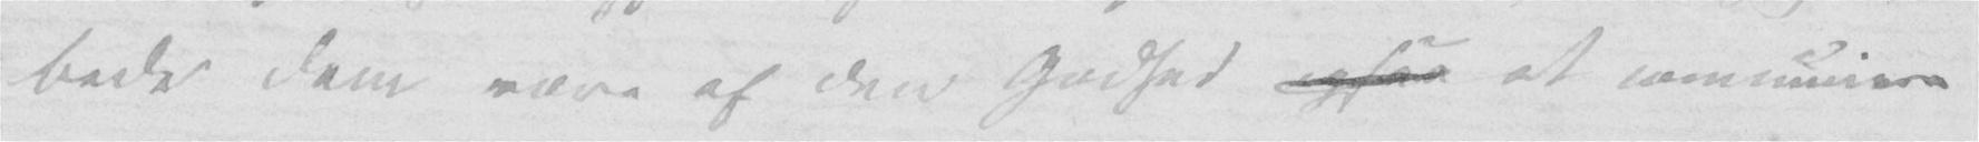bede Dem være af den Godhed ogsaa at communicere
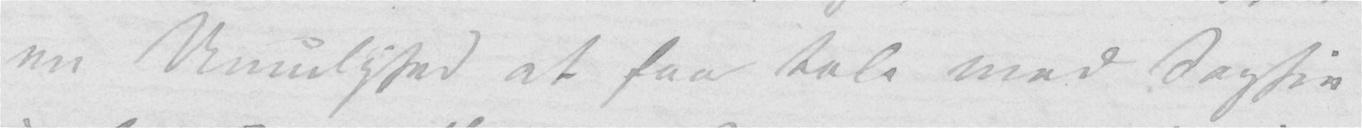en Umulighed at faa tale med Sophie
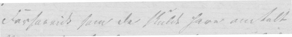Forsaavidt som De skulde have omtalt
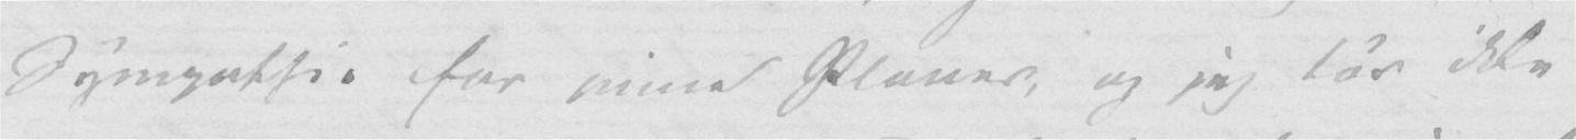Sympathie for mine Planer, og jeg tør ikke
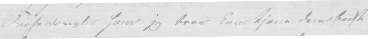Forholdsregler som jeg tror kan tjene dem bedst.
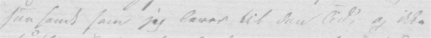saa sandt som jeg lever til den Tid, og ikke
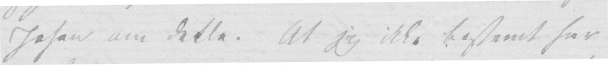Johan om dette. At jeg ikke bestemt har
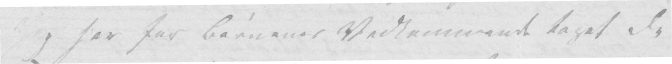Jeg har for Børnenes Vedkommende taget de
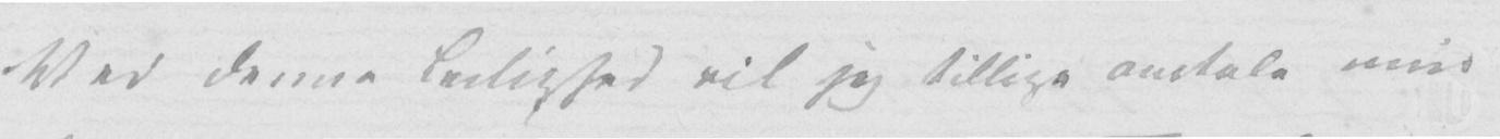Ved denne Leilighed vil jeg tillige omtale min
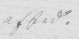afsted.
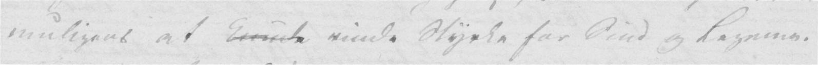muligens at kunde vinde Styrke for Sind og Legeme.
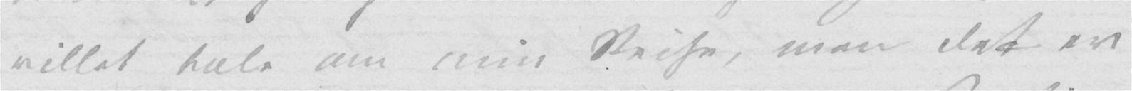villet tale om min Reise, men det er
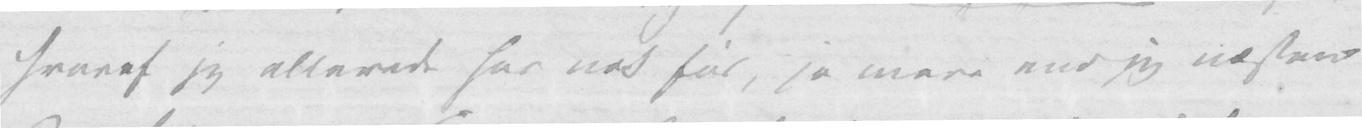hvoraf jeg allerede har nok før, ja mere end jeg næsten
